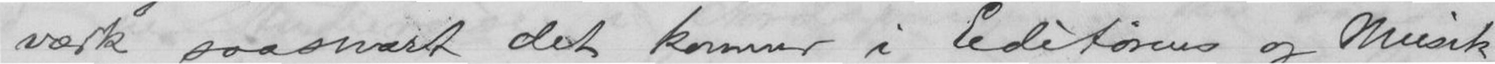værk saasnart det kommer i Editørens og Musik
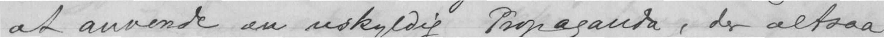at anvende en uskyldig Propaganda, der altsaa
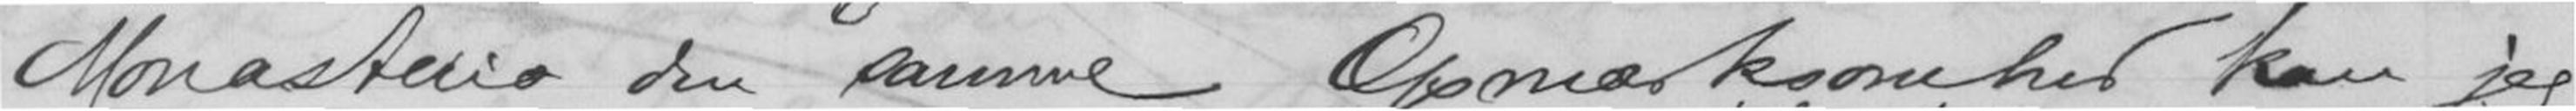Monasterio den samme Opmærksomhed kan jeg
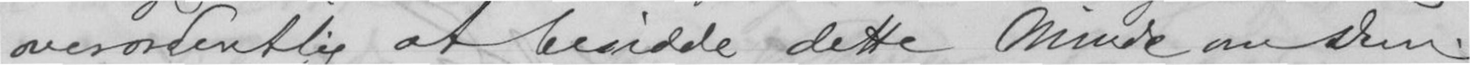overordentlig at besidde dette Minde om Dem.
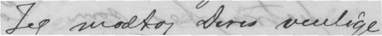Jeg modtog Deres venlige
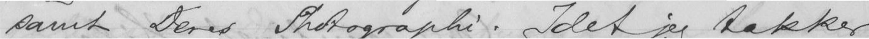samt Deres Photographi. Idet jeg takker
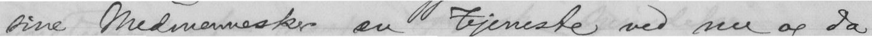sine Medmennesker en tjeneste ved nu og da
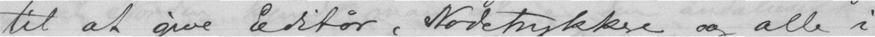til at give Editør, Nodetrykkere og alle i
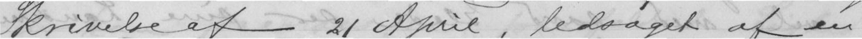Skrivelse af 21 April, ledsaget af en
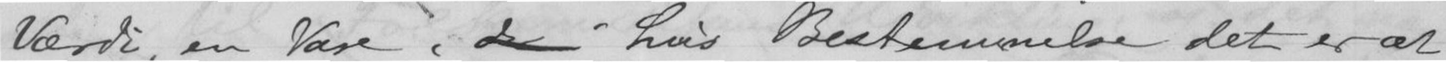Værdi, en Vare i de hvis Bestemmelse det er at
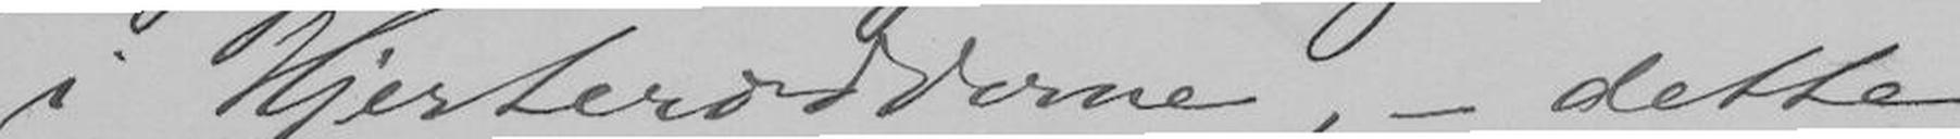i Hjerterødderne, - dette
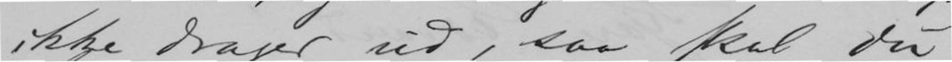ikke drager ud, saa skal Du
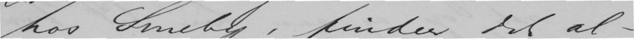hos Smeby, finder det al-
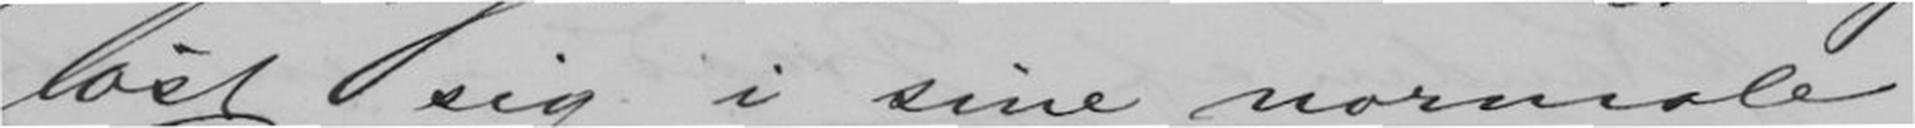løst sig i sine normale
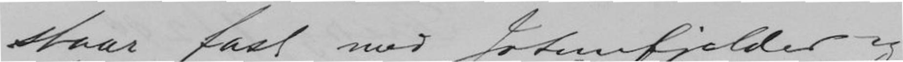staar fast med Jotunfjelder
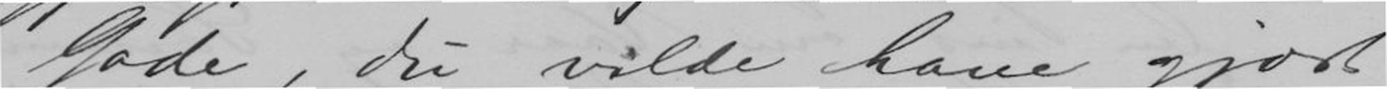Gode, Du vilde have gjort
